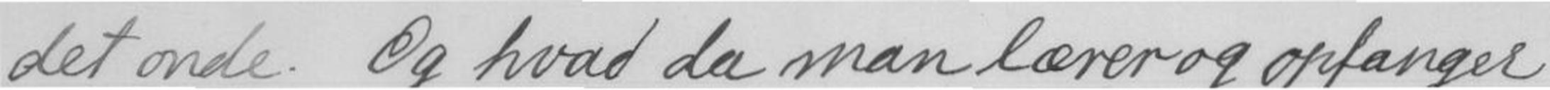det onde. Og hvad da man lærer og opfanger
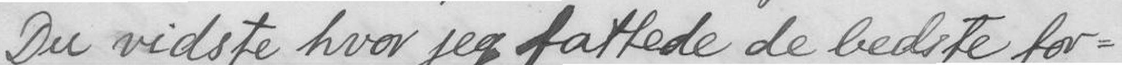Du vidste hvor jeg fattede de bedste for-
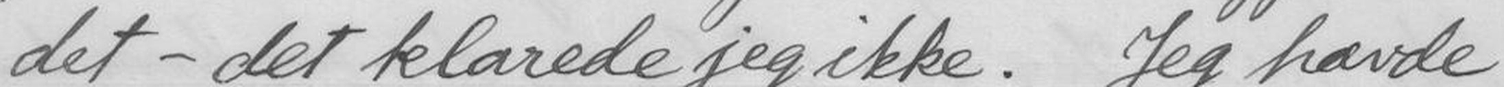det - det klarede jeg ikke. Jeg havde
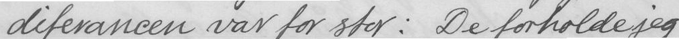diferancen var for stor: De forholde jeg
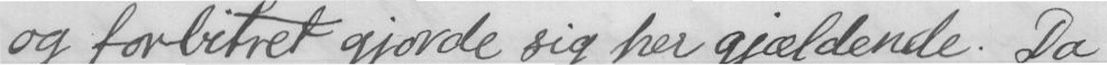og forbitret gjorde sig her gjældende. Da
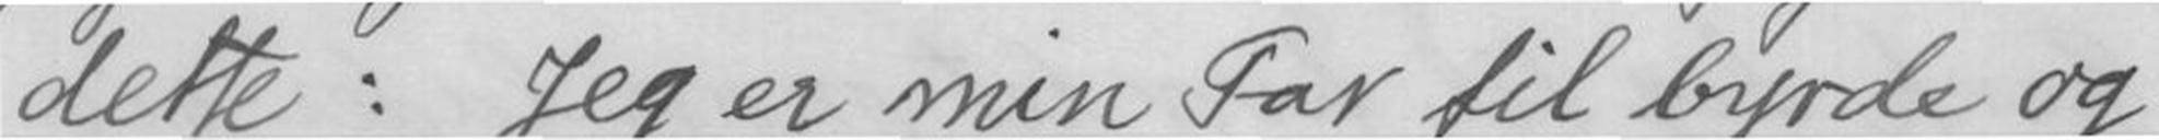dette: Jeg er min Far til byrde og
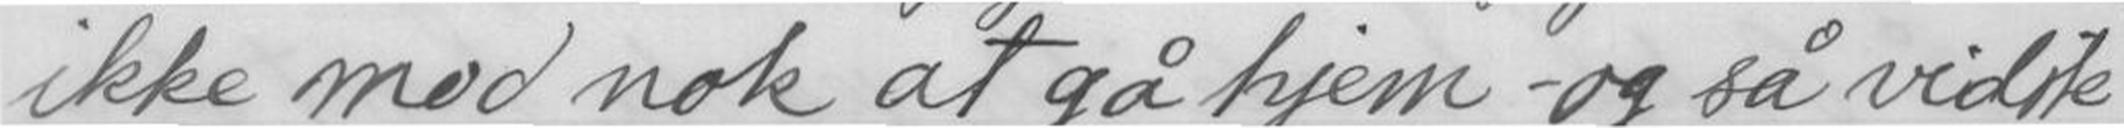ikke mod nok at gå hjem og så vidste
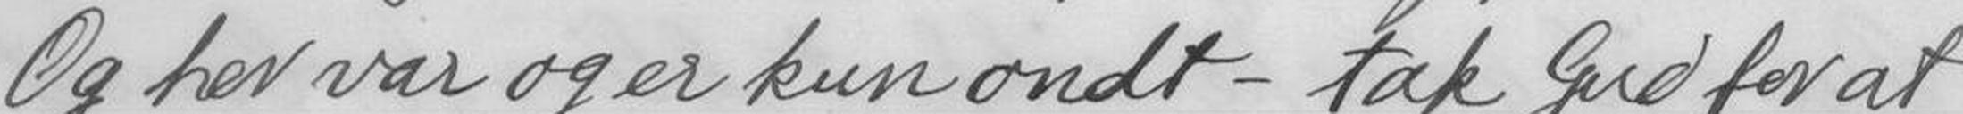Og her var og er kun ondt - tak Gud for at
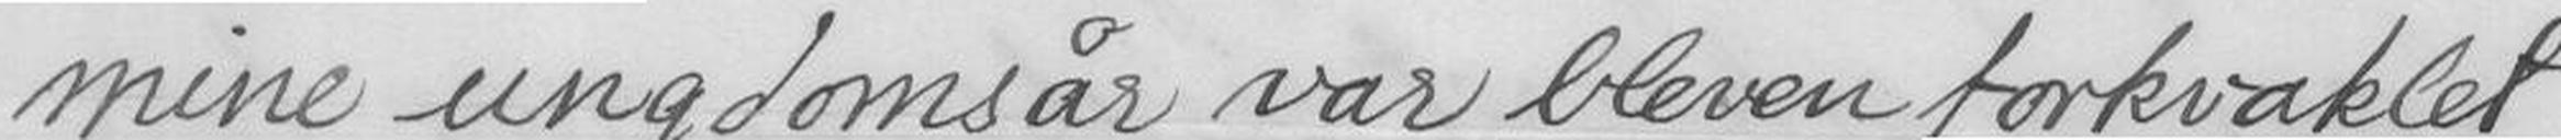mine ungdomsår var bleven forkvaklet
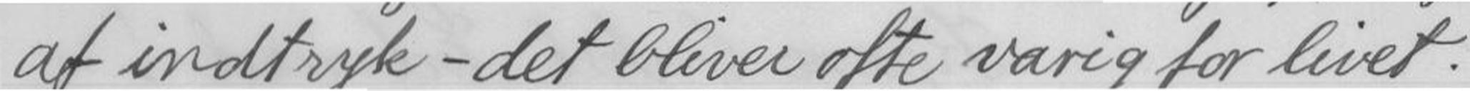af indtryk - det bliver ofte varig for livet.
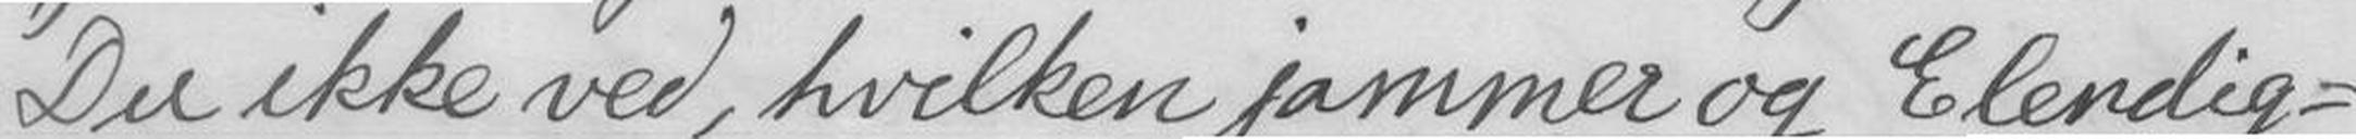Du ikke ved, hvilken jammer og Elendig-
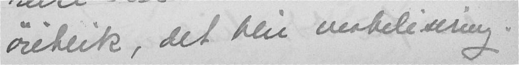øieblik, det blir mobelisering.
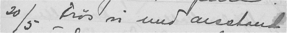20/5 Frøs vi med arsstand
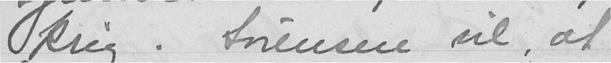krig. Sørensen vil, at
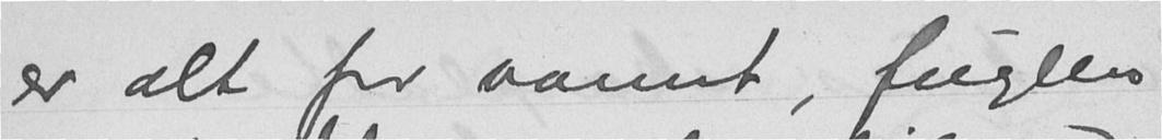er alt for varmt, fugler
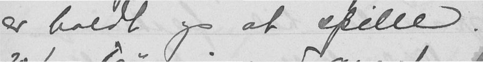er holdt op at spille.
